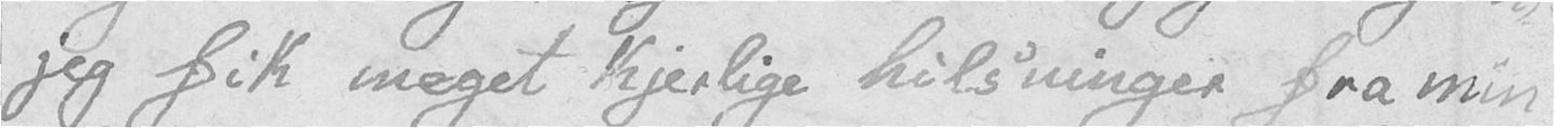jeg fik meget kjerlige hilsninger fra min
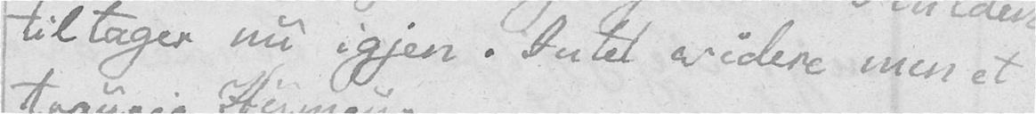tiltager nu igjen. Intet videre men et
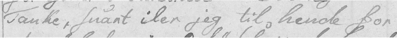Tanke, snart iler jeg til hende for
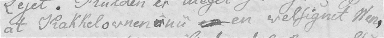at Kakkelovnen er nu en en velsignet Ven,
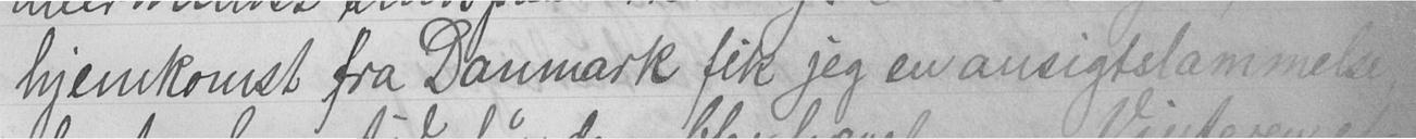hjemkomst fra Danmark fik jeg en ansigtslammelse
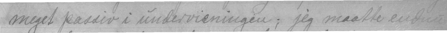meget passiv i undervisningen; jeg maatte endnu
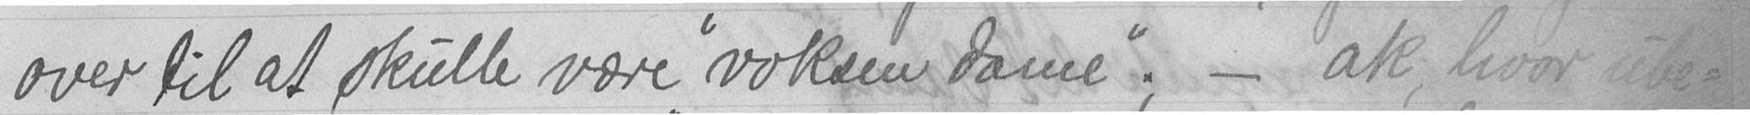over til at skulle være "voksen dame"; - ak, hvor ube-
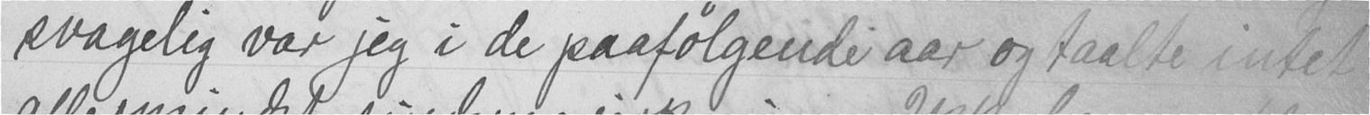svagelig var jeg i de paafølgende aar og taalte intet
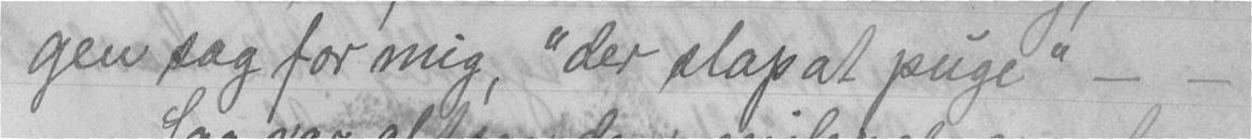gen sag for mig, "der slap at puge" - -
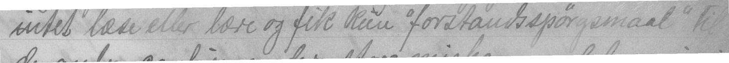intet læse eller lære og fik kun "forstandsspørgsmaal" til
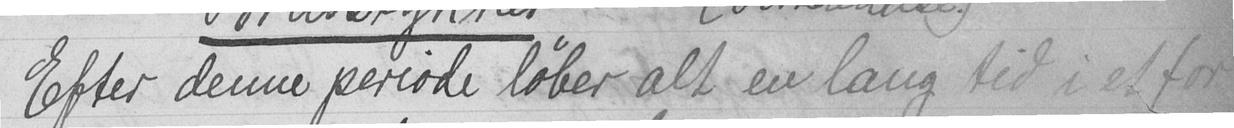Efter denne periode løber alt en lang tid i et for
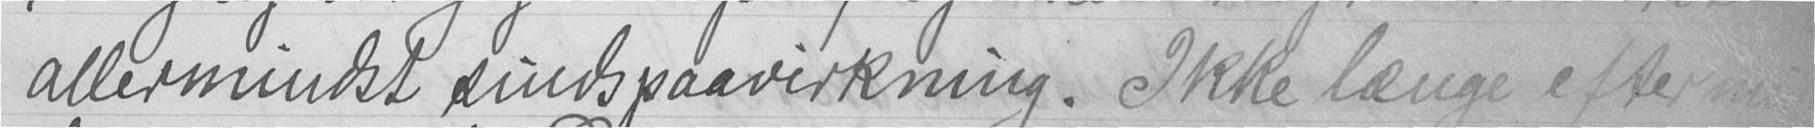allermindst sindspaavirkning. Ikke længe efter min
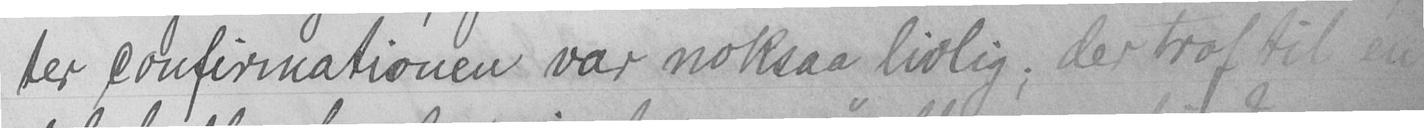ter confirmationen var noksaa livlig; der traf til en
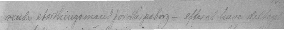rende storthingsmand for Sarpsborg - efter at have deltaget
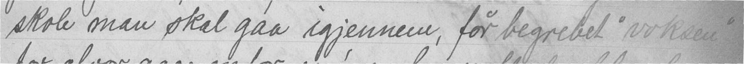skole man skal gaa igjennem, før begrebet "voksen"
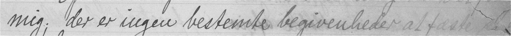mig; der er ingen bestemte begivenheder at fæste
